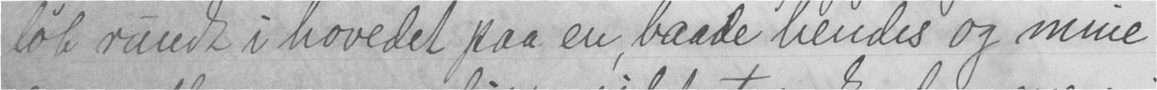løb rundt i hovedet paa en, baade hendes og mine
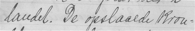landet. De opslaaede kron-
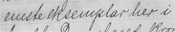eneste eksemplar her i
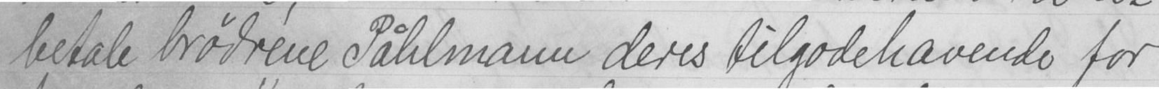betale brødrene Påhlmann deres tilgodehavende for
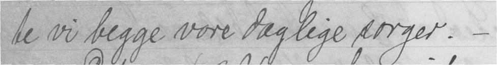te vi begge vore daglige sorger. -
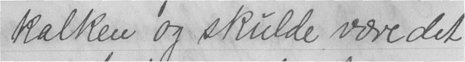kalken og skulde være det
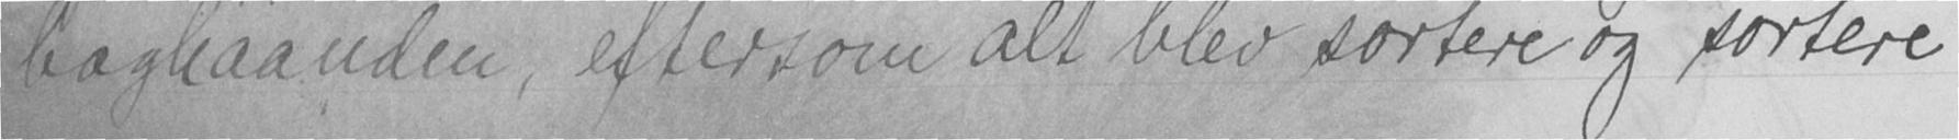baghaanden, eftersom alt blev sortere og sortere
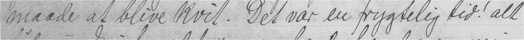maade at blive kvit. Det var en frygtelig tid! alt
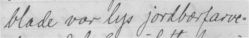blade var lys jordbærfarve-
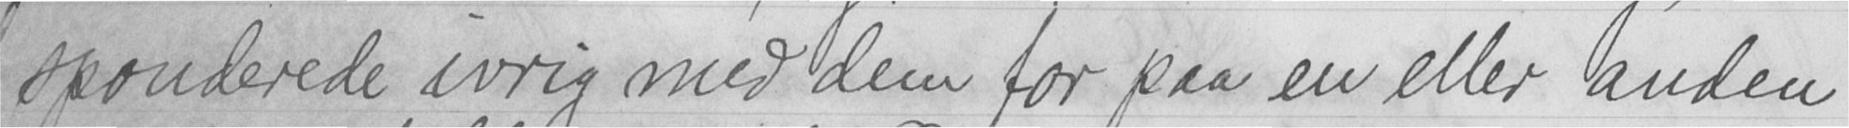sponderede ivrig med dem for paa en eller anden
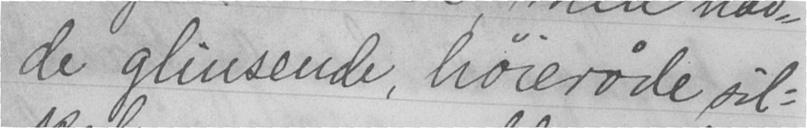de glinsende, høierøde sil-
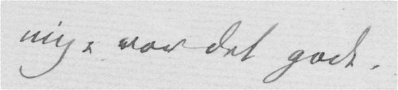mig, var det godt.
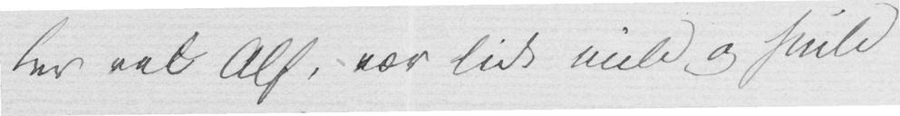Lev vel Alf, vær lidt mild og snild
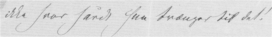ikke hvor hårdt hun trænger til det!
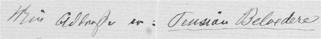Min Addresse er: Pension Belvedere
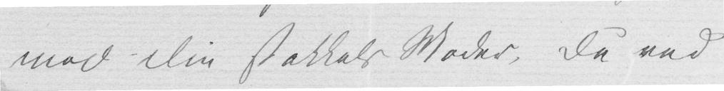mod Din stakkels Moder, Du ved
